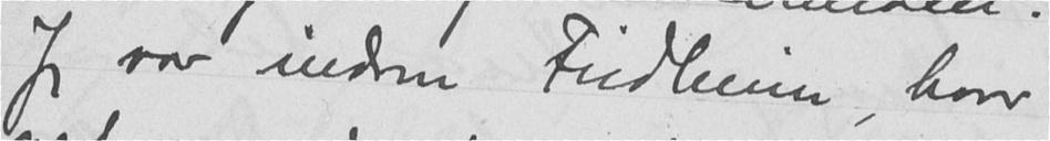Jeg var indom Fridheim, hvor
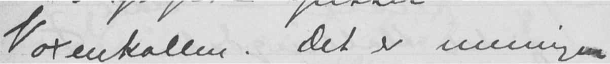Voxenkollen. Det er meningen
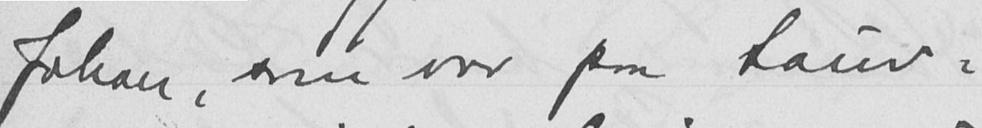Johan, som var paa Lauv-
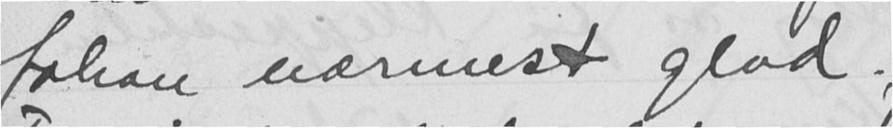Johan nærmest glad.
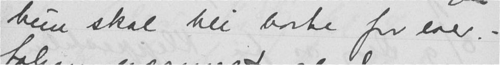hun skal bli borte for ever. -
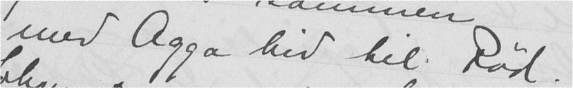med Agga hid til Rød.
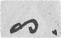os.
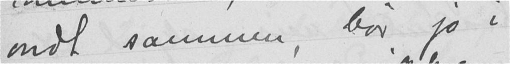ondt sammen, bør jo i
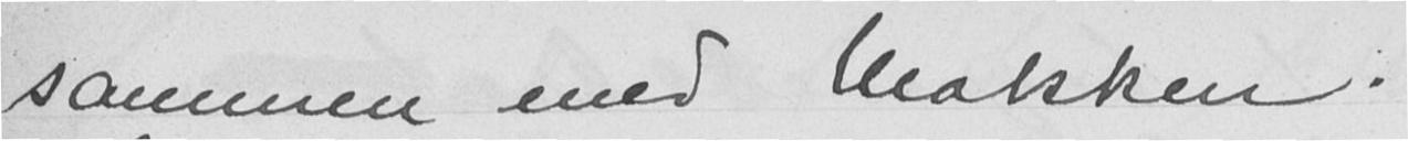sammen med Makken.
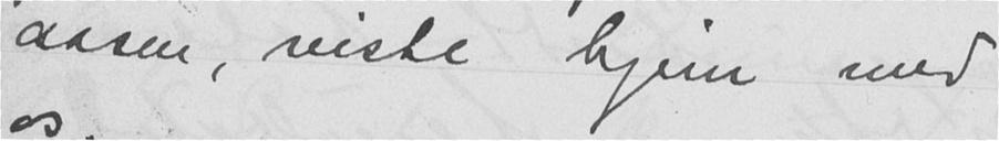aasen, reiste hjem med
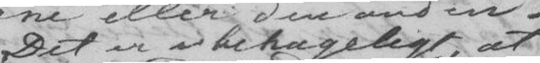Det er ubehageligt, at
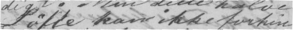Løfte kan ikke forhin
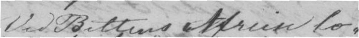Ved Bittens Afreise lo-
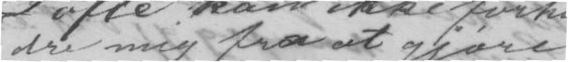dre mig fra at gjøre
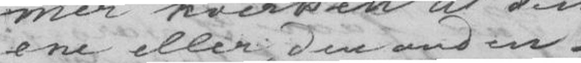ene eller Den anden.
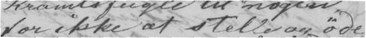for ikke at stelle og øde
